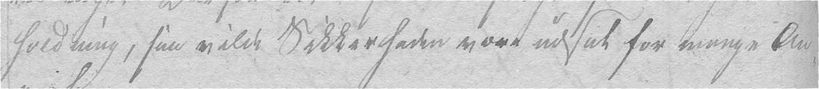holdning, saa vilde Sikkerheden være udsat for mange An-
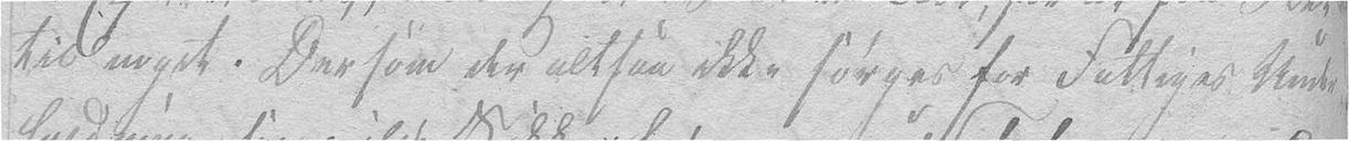til noget. Dersom der altsaa ikke sørges for Fattiges Under-
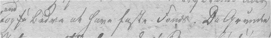-og bedre at have faste Fonds. De Grunde
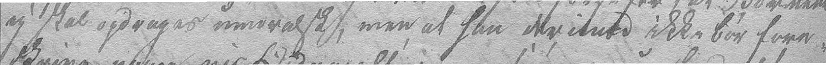ey skal opdrages umoralske, men at han derunder ikke bør fore-
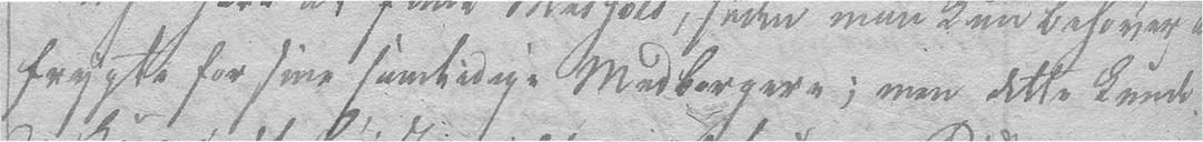frygte for sine samtidige Medborgere; men dette kunde
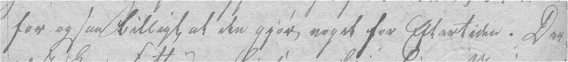for ogsaa billigt at den gjør noget for Eftertiden. Der-
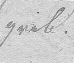greb.
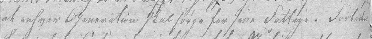at enhver Generation skal sørge for sine Fattige. Fortiden
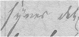synes det
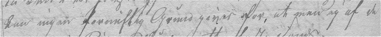kan ingen fornuftig Grund gives for, at man ey af de
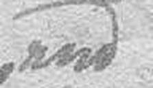end
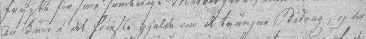da kun i det høieste gjelde om de tvungne Bidrag, og der
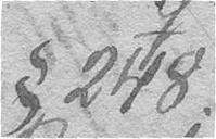§ 248.
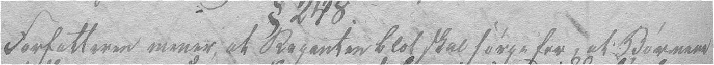Forfatteren mener, at Regenten blot skal sørge for, at Børnene
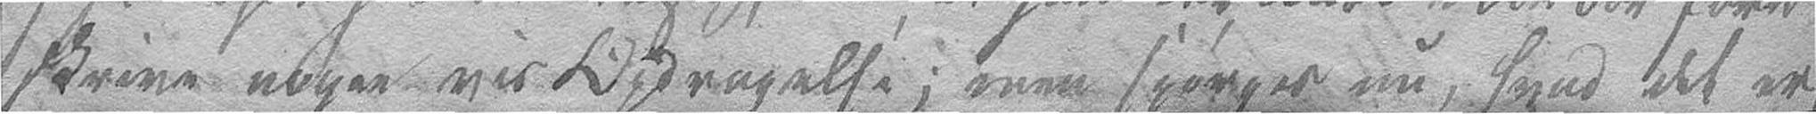skrive nogen vis Opdragelse; men spørges nu, hvad det er
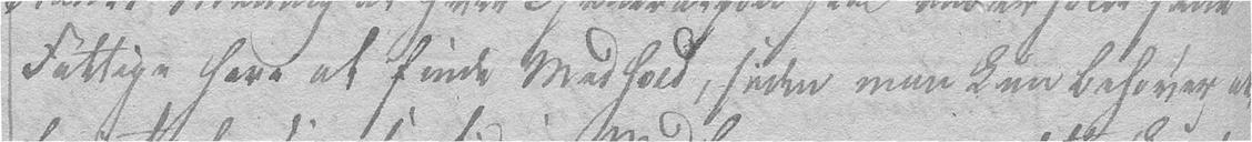Fattige heri at finde Medhold, siden man kun behøver at
